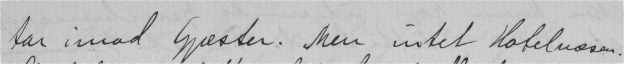tar imod Gjæster. Men intet Hotelvæsen.
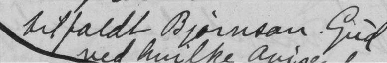tilfaldt Bjørnson. Gud
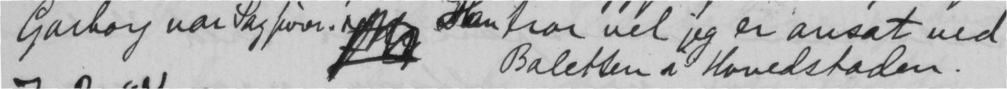Garborg var Sag fører;  Han tror vel jeg er ansat ved
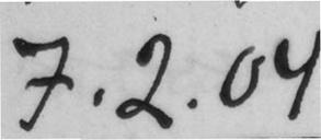7.2.04
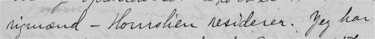rigmænd - Homslien residerer. Jeg har
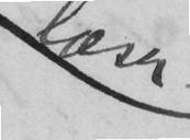læser
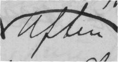aften
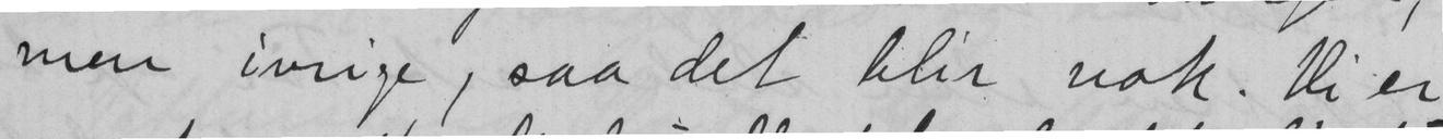men ivrige, saa det blir nok. Vi er
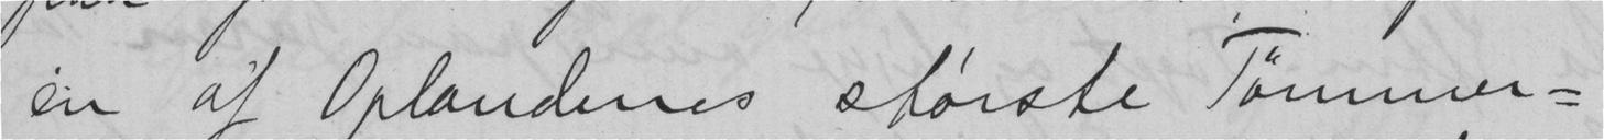en af Oplandenes største Tømmer-
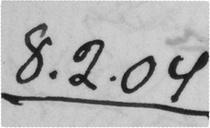8.2.04
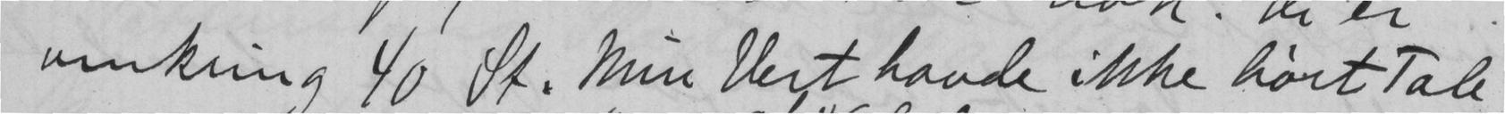omkring 40 St. Min Vert havde ikke hørt Tale
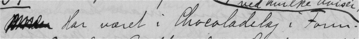Har været i Chocoladelag i Form.
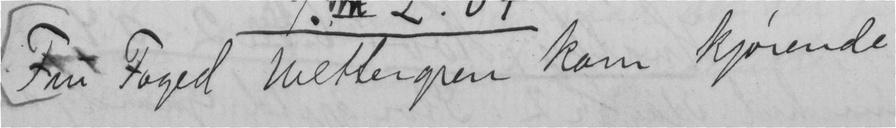Fru Foged Wettergren kom kjørende
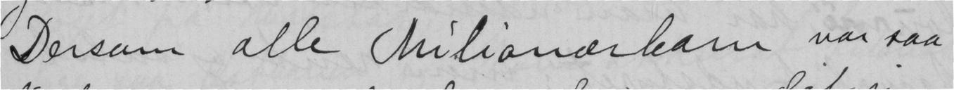Dersom alle Milionærbarn var saa
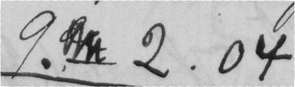9. 2.04
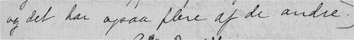og det har ogsaa flere af de andre.
